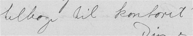tilbage til kontoret?
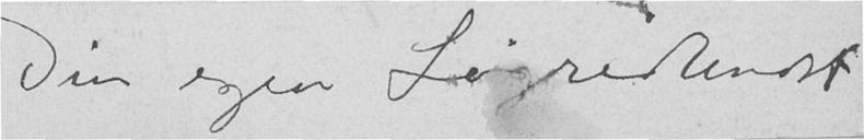Din egen Sigrid Undset
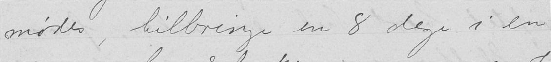mødes, tilbringe en 8 dage i en
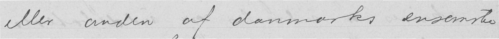eller anden af danmarks ensomste
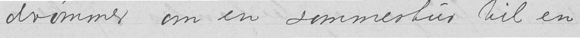drømmer om en sommertur til en
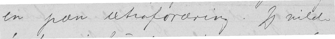en pen extraforæring. Jeg vilde
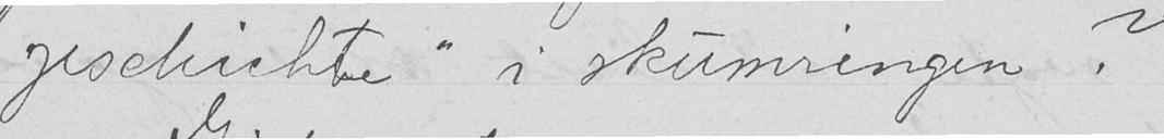geschichte" i skumringen?
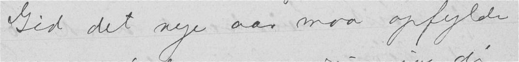Gid det nye aar maa opfylde
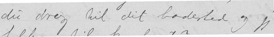du drog til dit badested og jeg
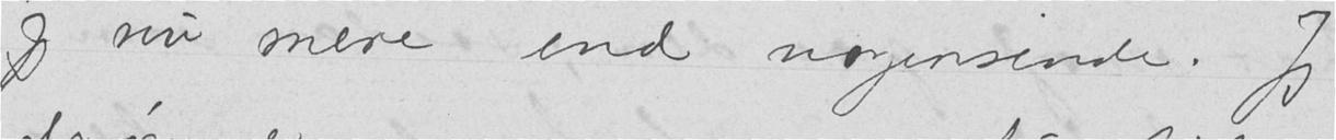jeg nu mere end nogensinde. Jeg
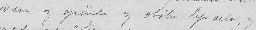væve og spinde og støbe lys selv, og
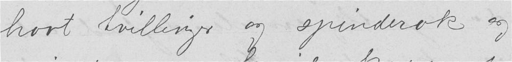havt tvillinger og spinderok og
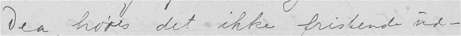Dea, høres det ikke fristende ud -
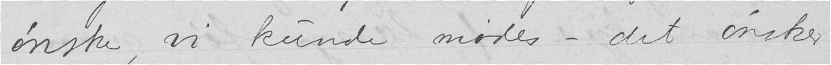ønske, vi kunde mødes - det ønsker
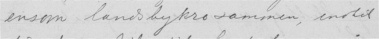ensom landsbykro sammen, indtil
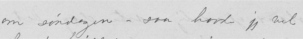om søndagen - saa havde jeg vel
